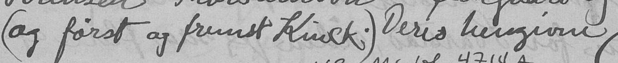(og først og fremst Kinck.) Deres hengivne,
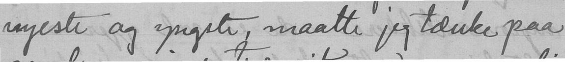nyeste - og yngste, maatte jeg tænke paa
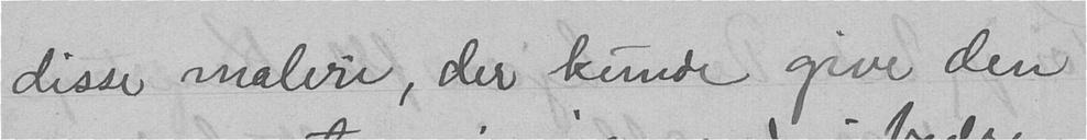disse maleri, der kunde give den
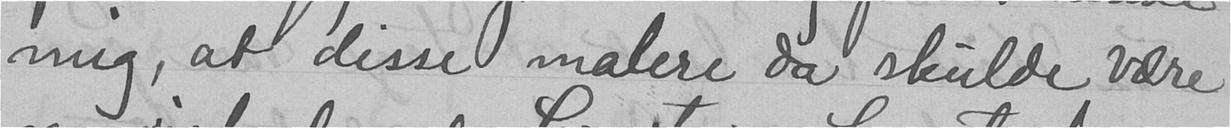mig, at disse malere da skulde være
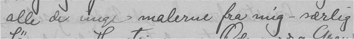alle de unge malerne fra mig - særlig
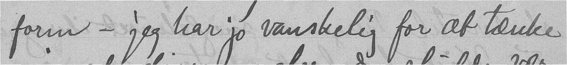form - jeg har jo vanskelig for at tænke
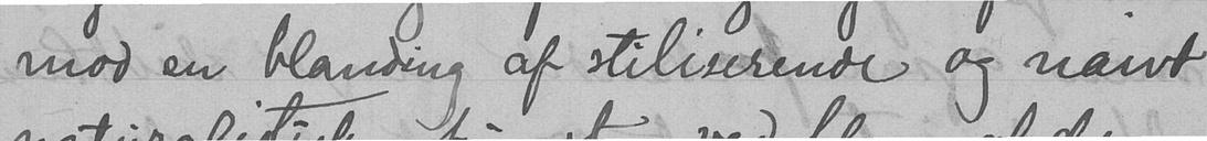mod en blanding af stiliserende - og naivt
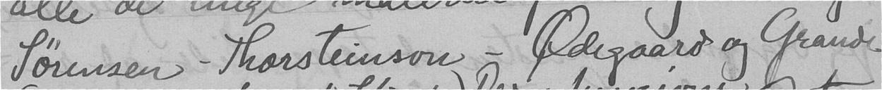Sørensen - Thorsteinson - Ødegaard og Grande
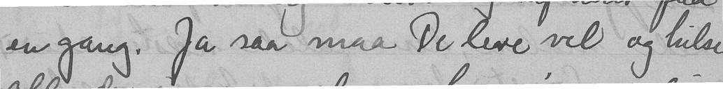en gang. Ja, saa maa De leve vel - og hilse
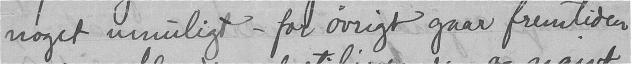noget umuligt - for øvrigt gaar fremtiden
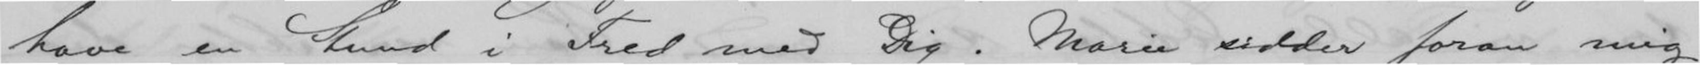have en Stund i Fred med Dig. Marie sidder foran mig
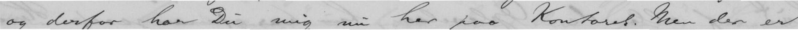og derfor har Du mig nu her paa Kontoret. Men der er
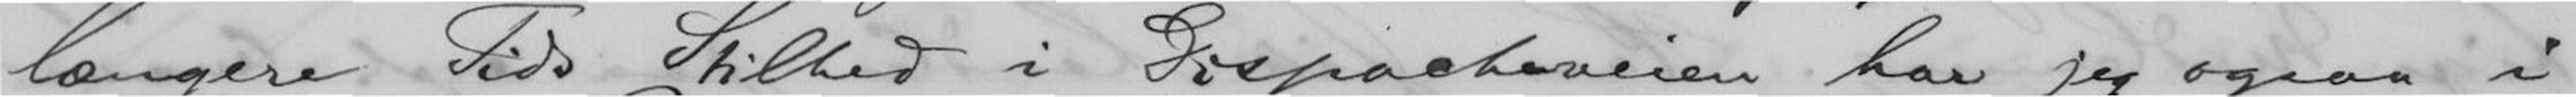længere Tids Stilhed i Dispacheveien har jeg ogsaa i
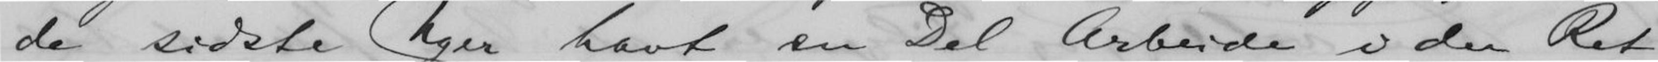de sidste Uger havt en Del Arbeide i den Ret-
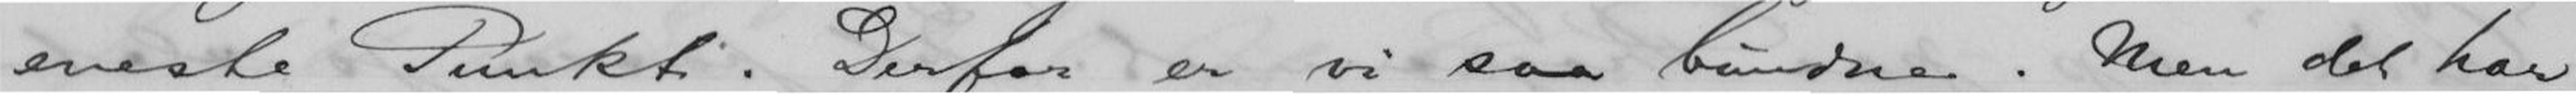eneste Punkt. Derfor er vi saa bundne. Men det har
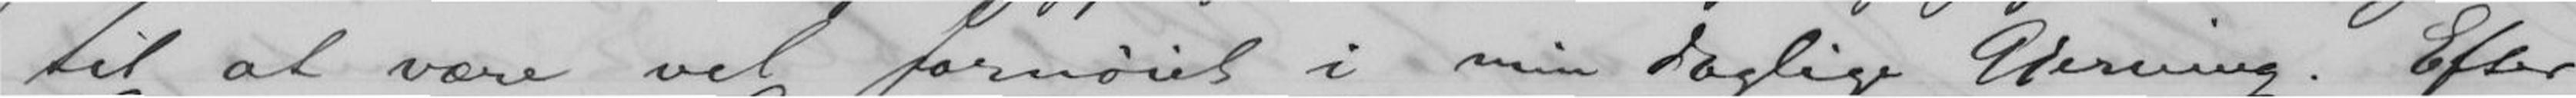til at være vel fornøiet i min daglige Gjerning. Efter
